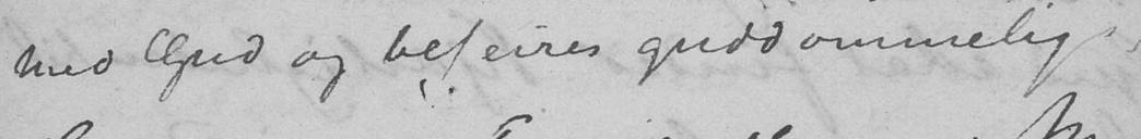med Gud og beseires guddommelig
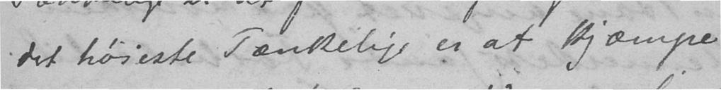det høieste Tænkelige er at kjæmpe
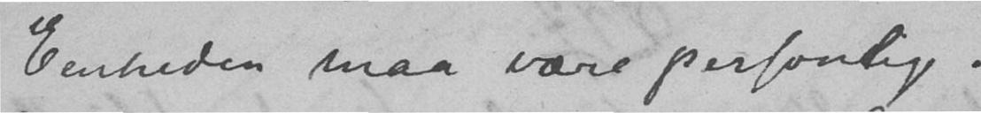Eenheden maa være personlig.
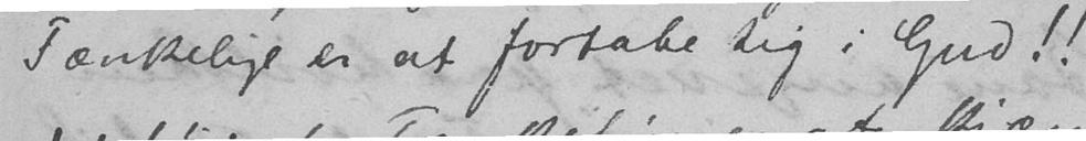Tænkelige er at fortale sig i Gud!!
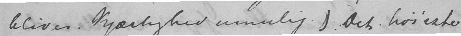bliver Kjærlighed umulig) Det høieste
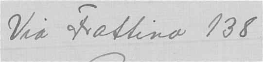Via Frattina 138
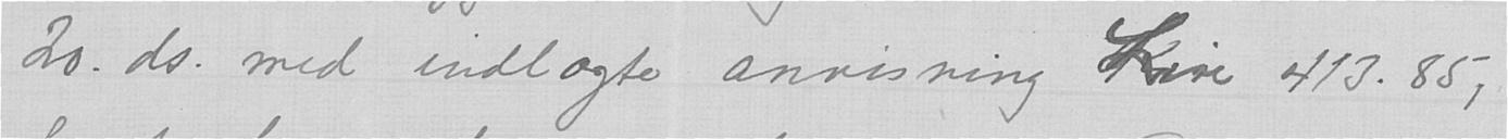20. ds. med indlagte anvisning Kr 413.85,
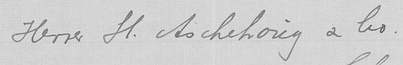Herrer H. Aschehoug & Co.
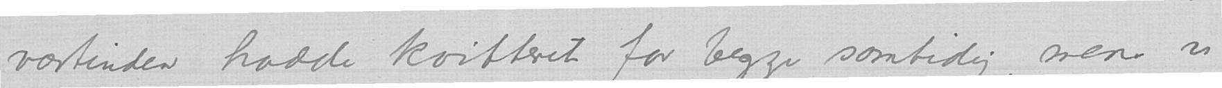værtinden hadde kvitteret for begge samtidig, mens vi
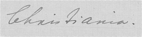Christiania.
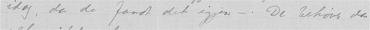idag, da de fandt det igjen –. De behøver da
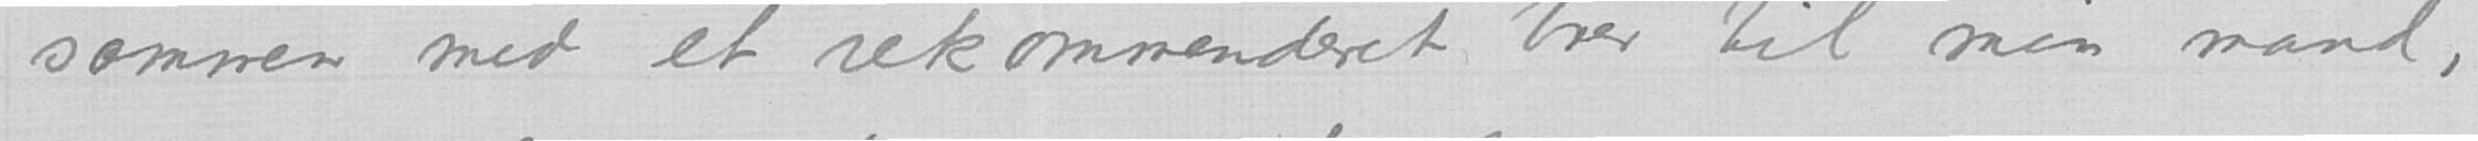sammen med et rekommenderet brev til min mand,
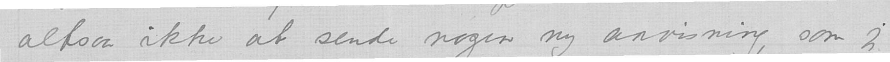altsaa ikke at sende nogen ny anvisning, som jeg
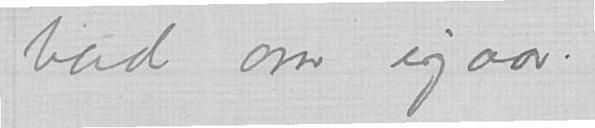bad om igaar.
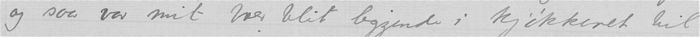og saa var mit brev blit liggende i kjøkkenet til
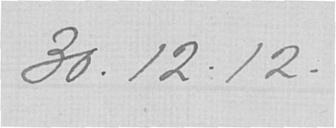30.12.12.
